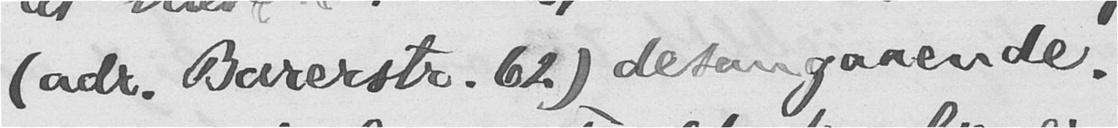(adr. Barerstr. 62) desangaaende.
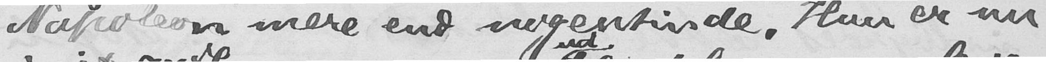Napoleon mere end nogensinde. Hun er nu
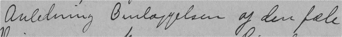Anledning Omlæggelsen af den fæle
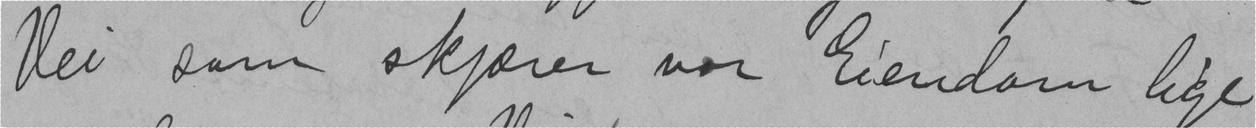Vei som skjærer vor Eiendom lige
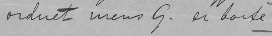ordnet mens G. er borte
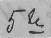5te
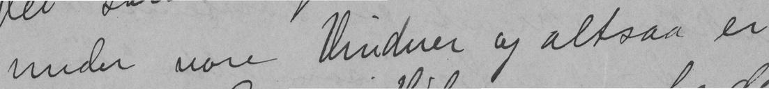under vore Vinduer og altsaa er
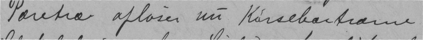Pæretræ afløser nu Kirsebærtrærne.
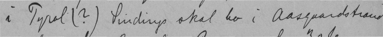i Tyrol (?) Sindings skal bo i Aasgaardstrand
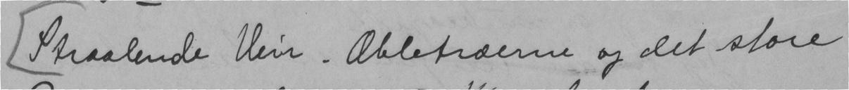Straalende Veir. Æbletræerne og det store
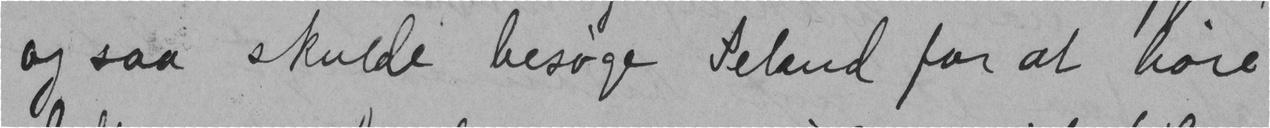og saa skulde besøge Seland for at høre
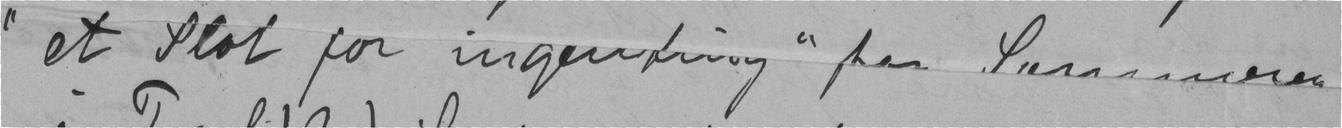"et Slot for ingenting" for Sommeren
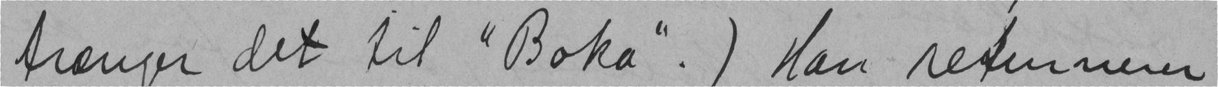trænger det til "Boka".) Han returnerer
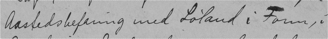Aastedsbefaring med Løland i Form, i
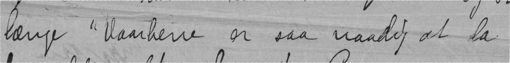længe "Vaarbene er saa naadig at la
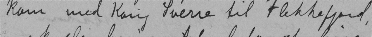kom med Kong Sverre til Flekkefjord,
